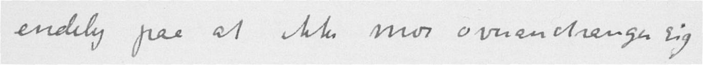endelig paa at ikke mor overanstrænger sig
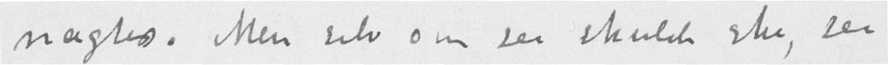nægtes. Men selv om saa skulde ske, saa
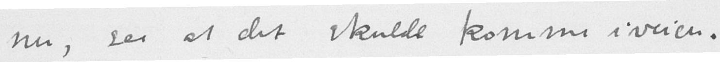nu, saa at det skulde komme i veien.
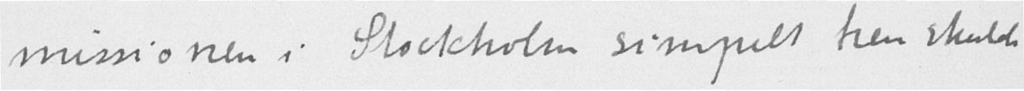missionen i Stockholm simpelt hen skulde
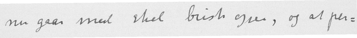nu gaar med skal briste ogsaa, og at per-
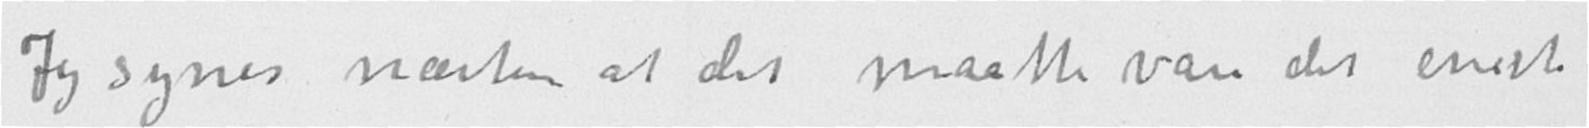Jeg synes næsten at det maatte være det eneste
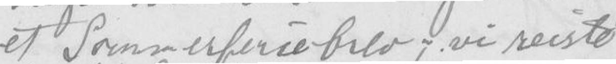et Sommerferiebrev; vi reiste
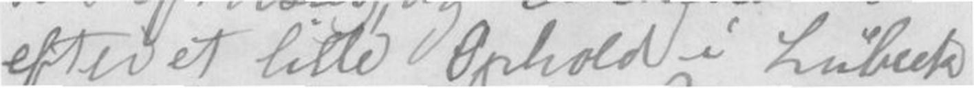efter et lille Ophold i Lübeck
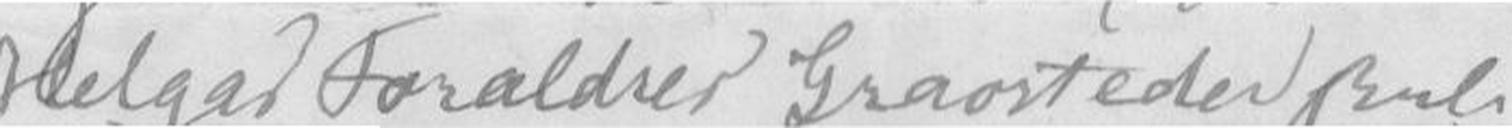Helgas Forældrer Graosteder?? skul-
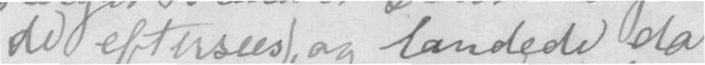de eftersees), og landede da
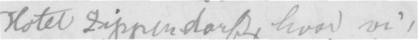Hotel Zippendorfs, hvor vi,
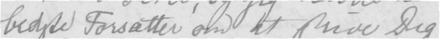bedste Forsætter om at skrive Dig
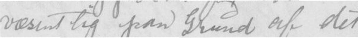væsentlig paa Grund af det
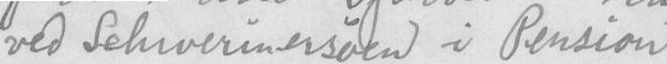ved Schwerinersøen i Pension
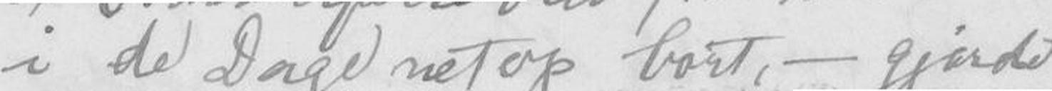i de Dage netop bort, - gjorde
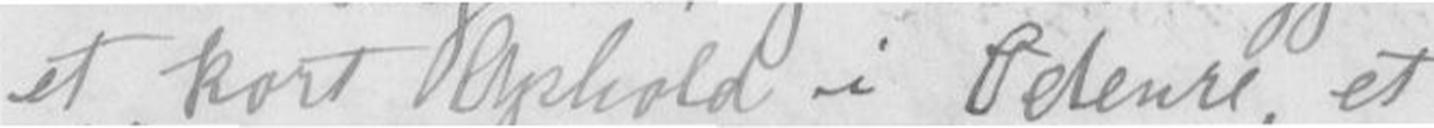et kort Ophold i Petenre??, et
